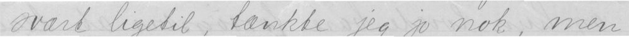svært ligetil, tænkte jeg jo nok, men
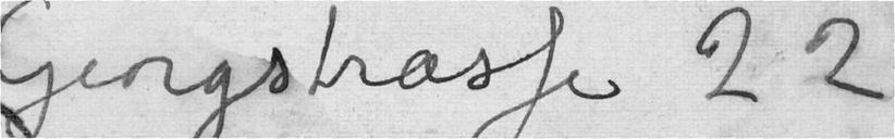Georgstrasse 22
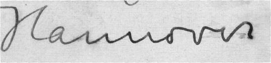Hannover
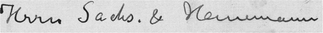Hrrn Sachse & Heinemann
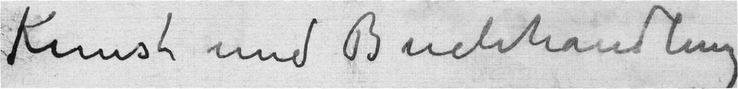Kunst und Buchhandlung
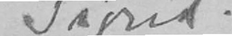Sigrid.
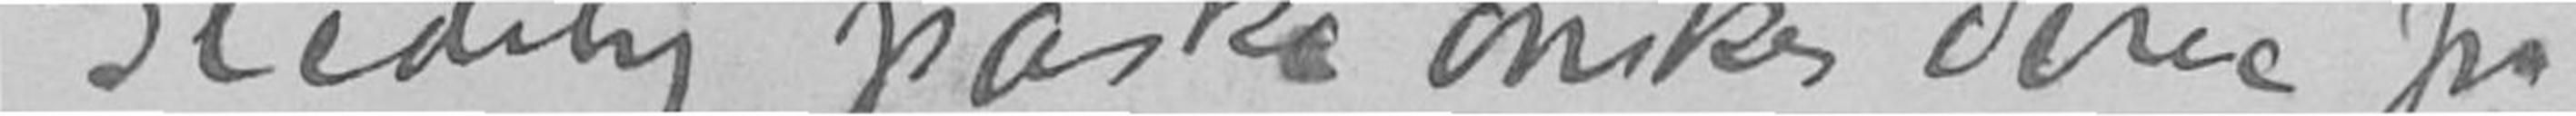Gledelig påske ønskes dere fra
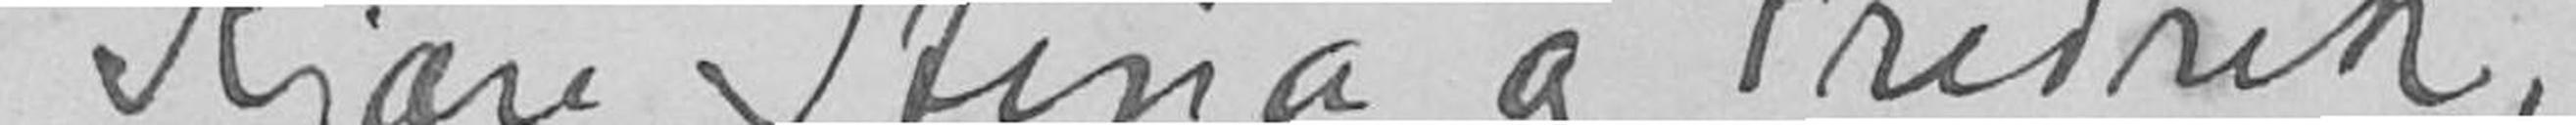Kjære Stina og Fredrik,
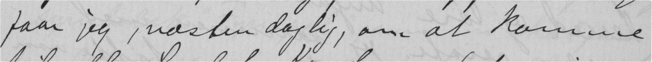faar jeg, næsten daglig, om at komme
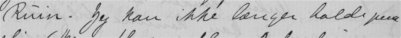Ruin. Jeg kan ikke længer holde paa
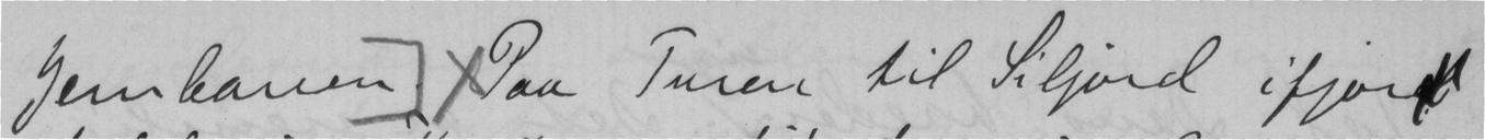Jernbanen. Paa Turen til Siljord ifjor
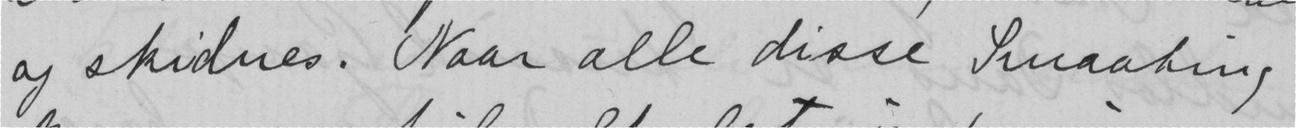og skidnes. Naar alle disse Smaating
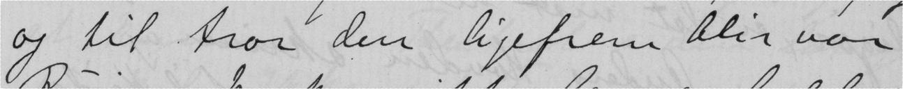og til tror den ligefrem blir vor
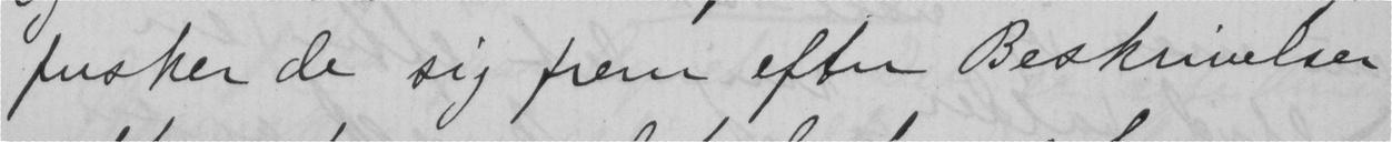fusker de sig frem efter Beskrivelser
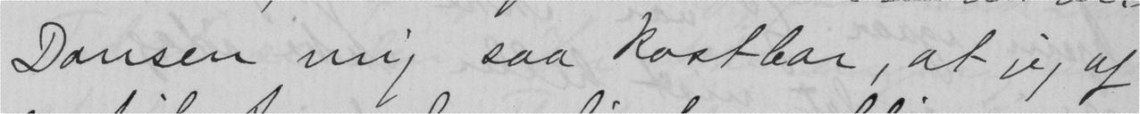Dansen mig saa kostbar, at jeg af
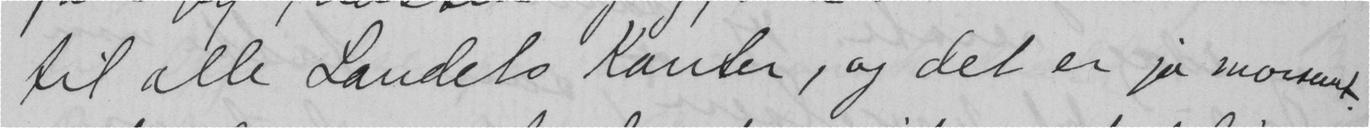til alle Landets Kanter, og det er jo morsomt.
